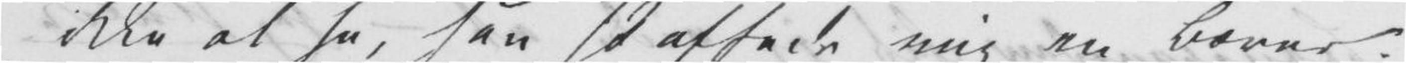ikke at se, han skaffede mig en Bærer.
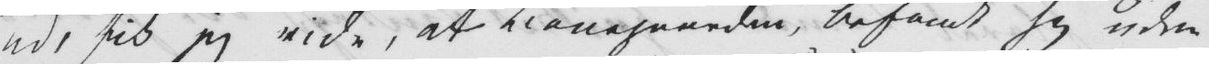ud, fik jeg vide, at Banegaarden, befandt sig uden
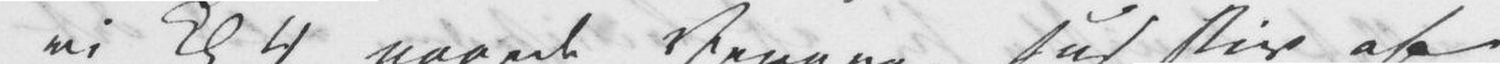vi Kl 4 naaede Verona, sad stiv af
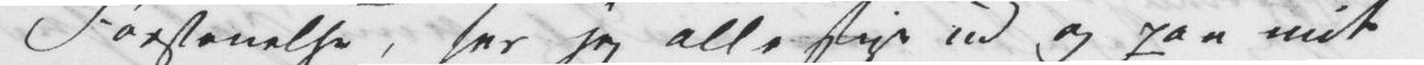Forstenelse, ser jeg alle stige ud og paa mit
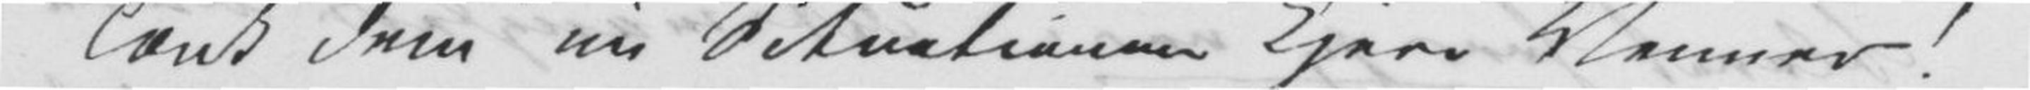Tænk Dem nu Situationen kjere Venner!
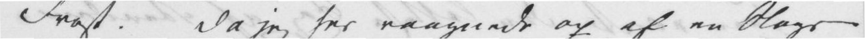Frost. Da jeg her vaagnede op af en Slags
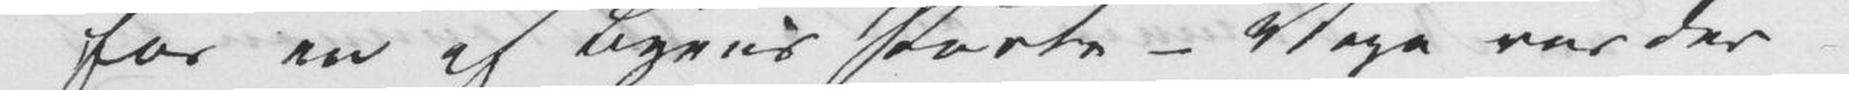for en af Byens Porte – Vogn var der
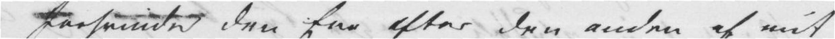forsvinder den Ene efter den anden af mit
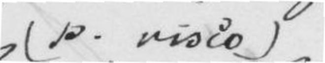<gap /></add>
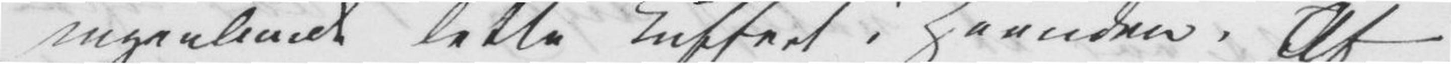ingenlunde lette Kuffert i Haanden. Af
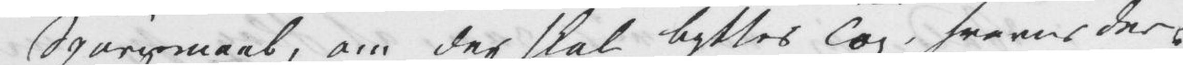Spørgsmaal, om der skal byttes Tog, svares der
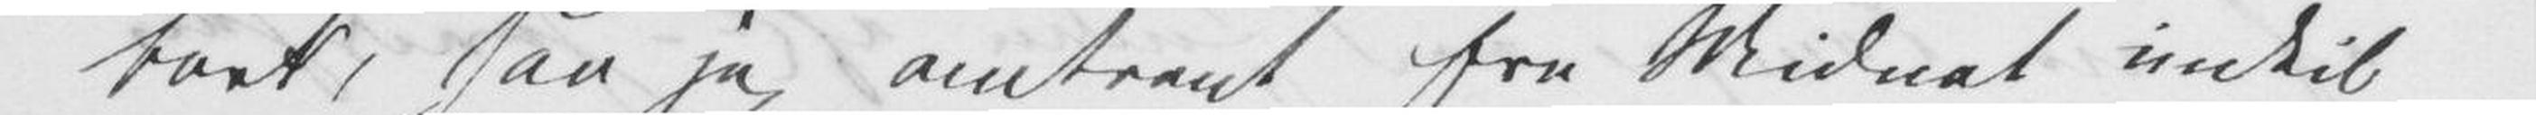bort, saa jeg omtrent fra Midnat indtil
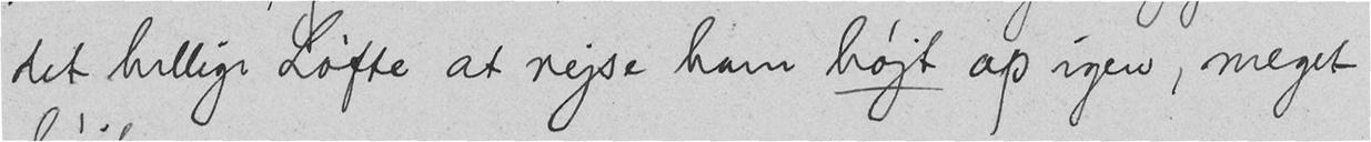det hellige Løfte at rejse ham højt op igen, meget
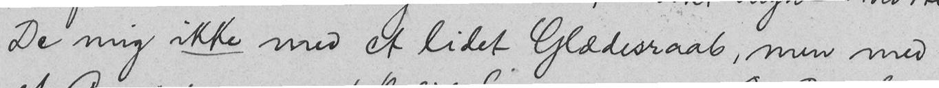De mig ikke med et lidet Glædesraab, men med
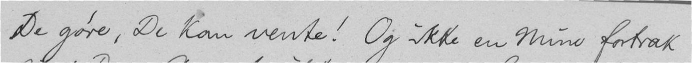De gøre, De kan vente! Og ikke en Mine fortrak
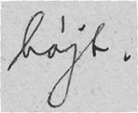højt.
